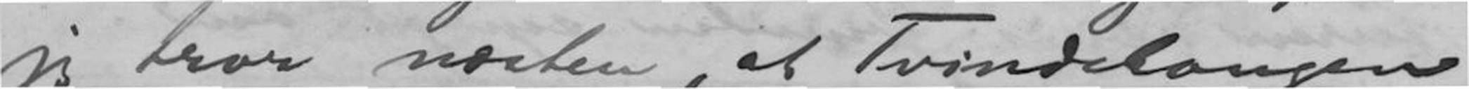jeg tror næsten, at Tvindehougen
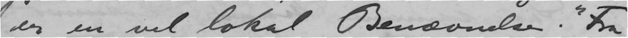er en vel lokal Benævnelse. "Fra
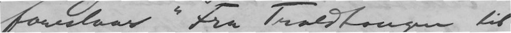foreslaar "Fra Troldhougen til
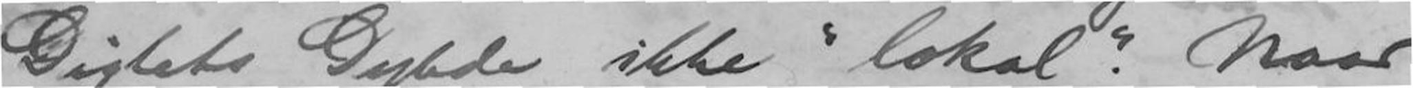Digtets Dybde ikke "lokal". Naar
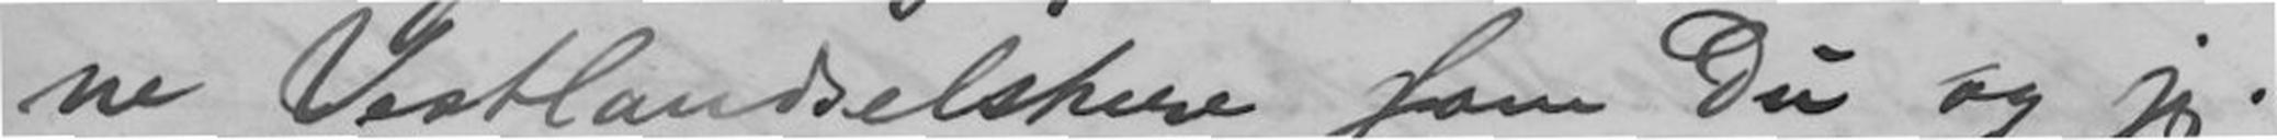ne Vestlandselskere som Du og jeg.
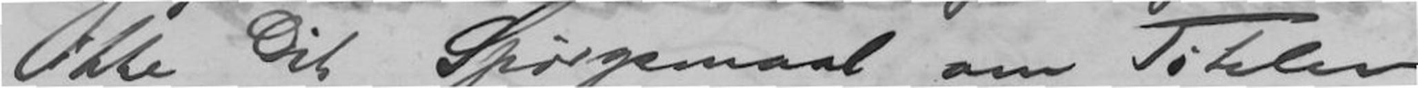ikke Dit Spørgsmaal om Titelen
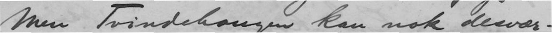Men Tvindehougen kan nok desvær-
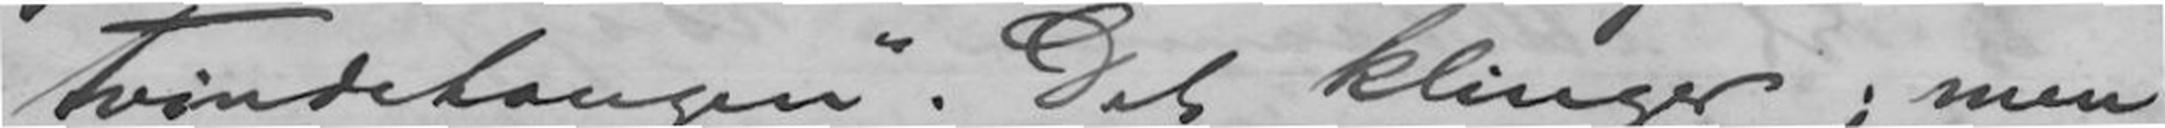Tvindehougen". Det klinger; men
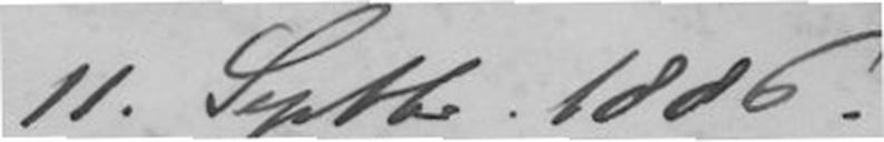11 Septbr. 1886.
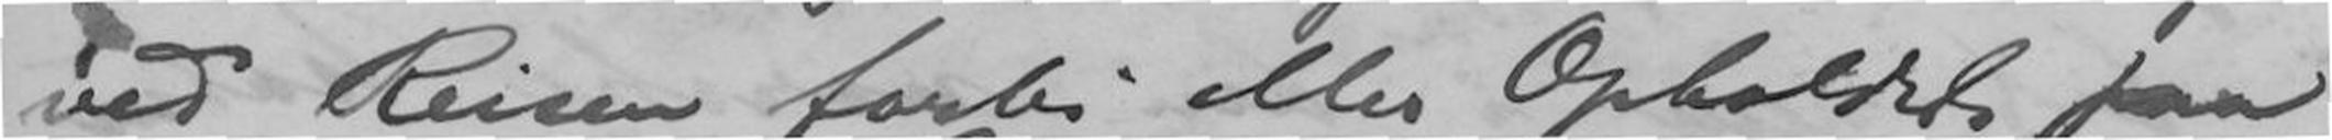ved Reisen forbi elles Opholdet paa
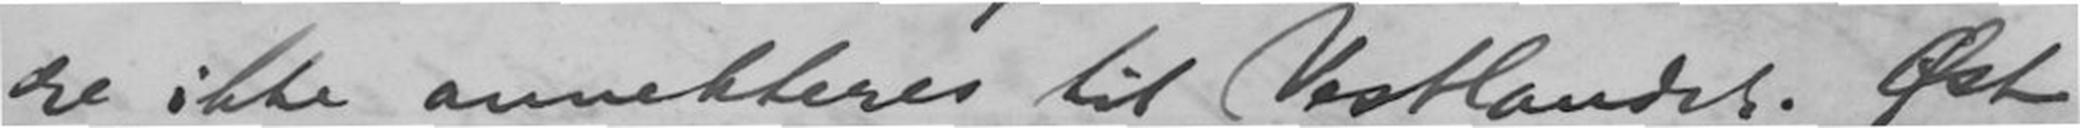re ikke annekteres til Vestlandet. Øst-
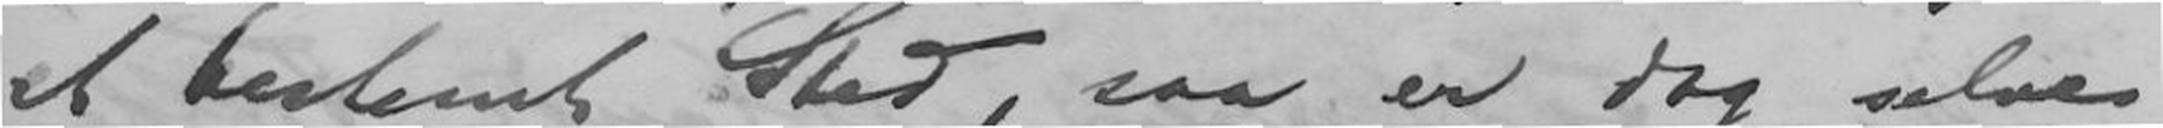et bestemt Sted, saa er dog selve
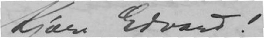Kjære Edvard!
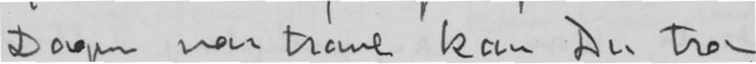Dagen var travl kan du tro.
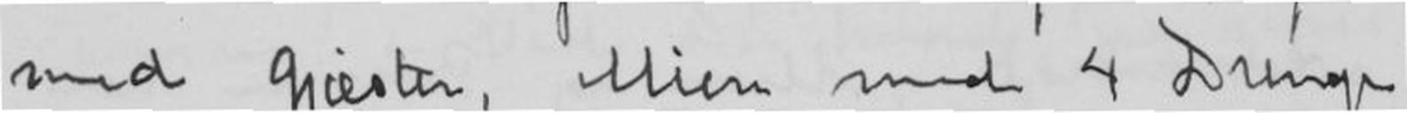med gjæster, Mien med 4 Drenger
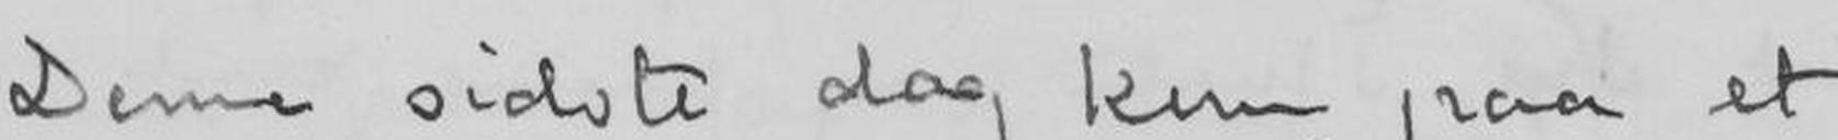Denne sidste dag kun paa et
